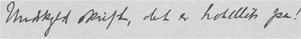Undskyld skriften, det er hotellets pen!
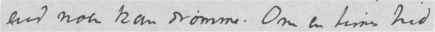end noen kan drømme.  Om en times tid
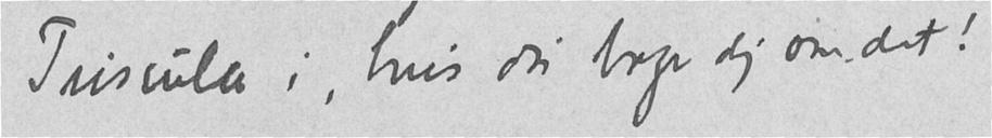Triscula i, hvis du bryr dig om det!
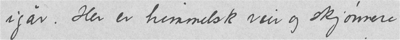igår.  Her er himmelsk veir og skjønnere
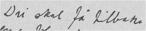Du skal få tilbake
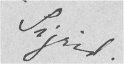Sigrid.
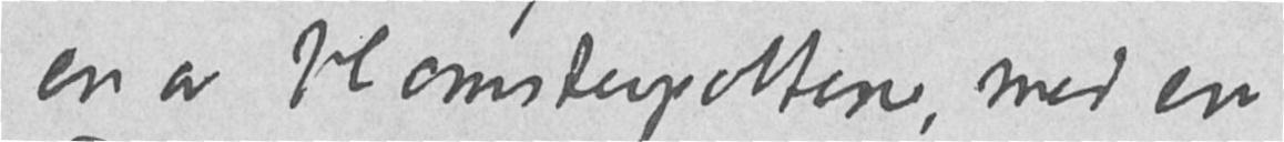en av blomsterpottene, med en
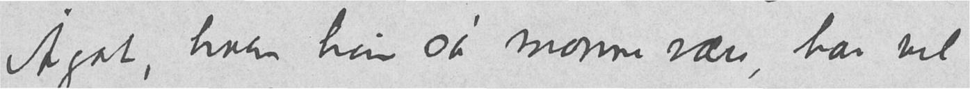Ågot, hvor hun så monne være, har vel
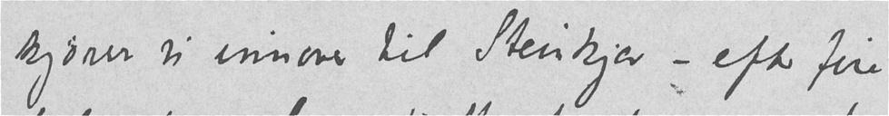kjører vi innom til Steinkjer - efter fire
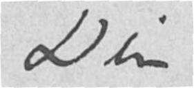Din
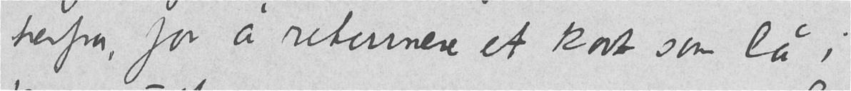herfra, for å returnere et kort som lå i
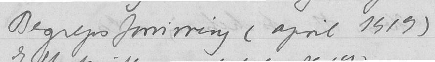Begrepsforvirring (april 1919)
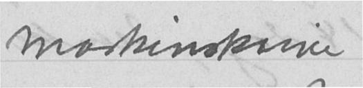maskinskrive
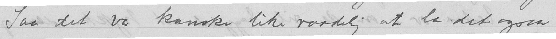Saa det var kanske like raadelig at la det ogsaa
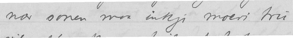nor sonen maa inkje moeri tru
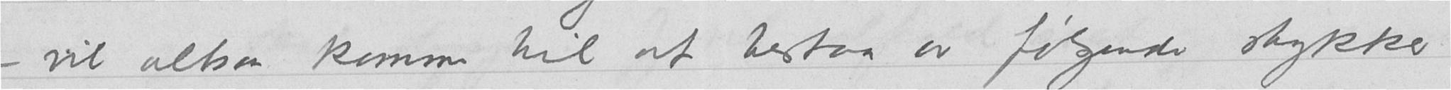– vil altsaa komme til at bestaa av følgende stykker
